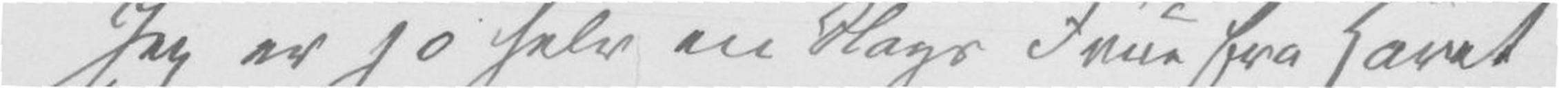Jeg er jo selv en Slags «Frue fra Havet»
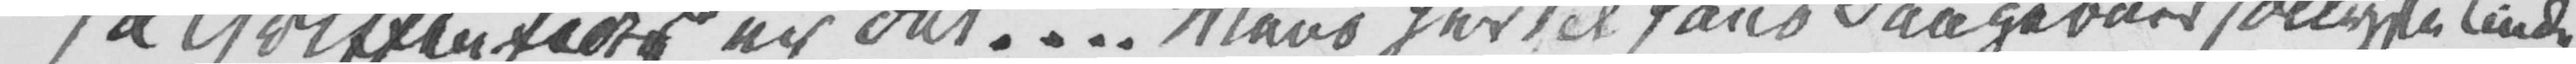Ja Griffenfe[l]dts er det! ... Mens her til hans Fangeburs sollyste Tinde
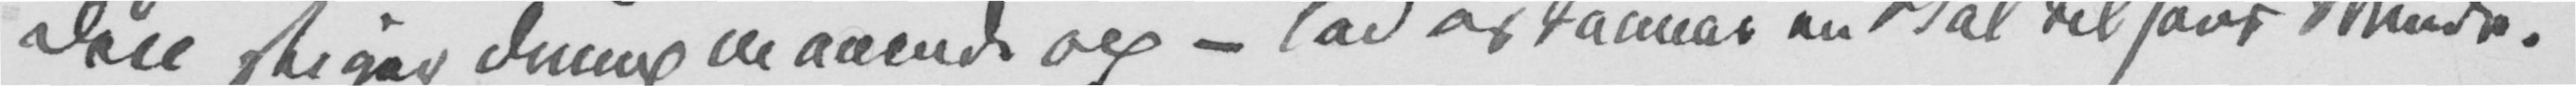Den stiger dump manende op – lad os tømme en Skål til hans Minde!
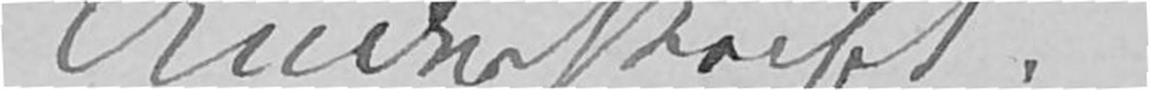Underskrift.
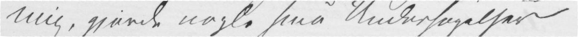mig, gjorde nogle små Undersøgelser
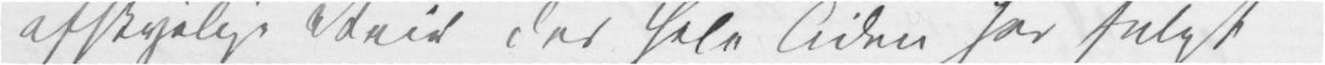afskyelige Veir der hele Tiden har fulgt
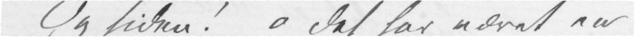Og siden! o det har været en
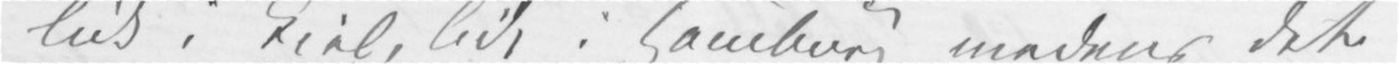lidt i Kiel, lidt i Hamburg medens det
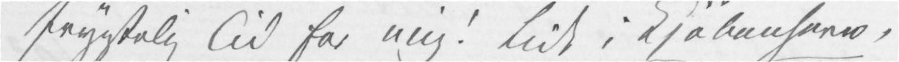frygtelig Tid for mig! Lidt i Kjøbenhavn,
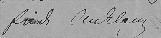finde Anklang.
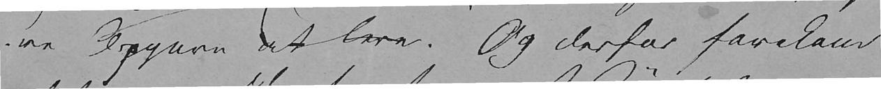Opgave at leve. Og derfor forekom
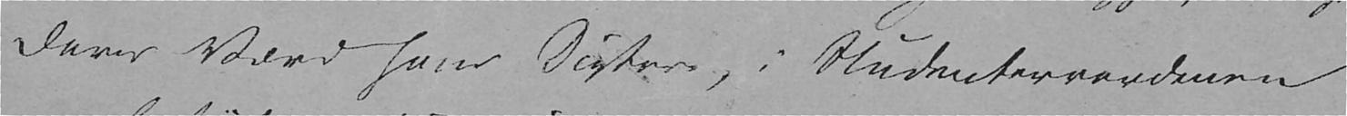deres Værd som Digtere, i Studenterverdenen
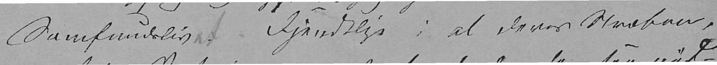Samfundsliv. Fjendtlige i al deres Stræben,
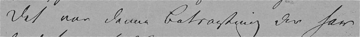Det var denne Betragtning der har
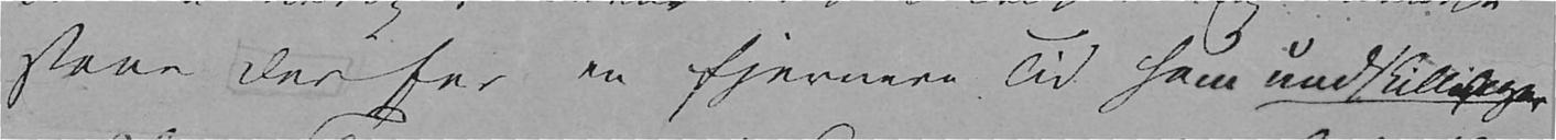staae der for en fjernere Tid som Uadskillelige.
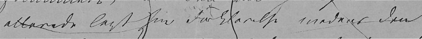allerede lagt sin Forklarelse medens den
